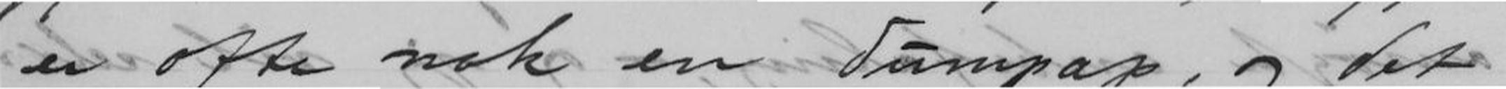er ofte nok en dumpap, og det
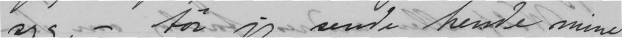syg, - tør jeg sende hende mine
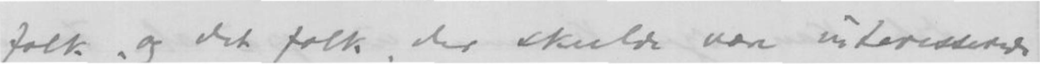folk, og det folk der skulde være interesseret,
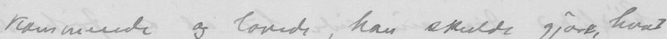kommende og lovede, han skulde gjøre hvad
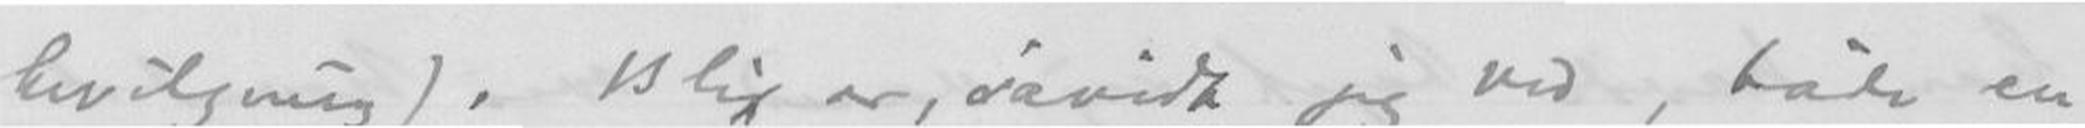bevilgning). Blix er, såvidt jeg ved, både en
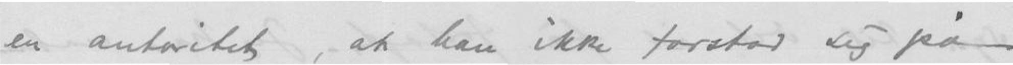en autoritet, at han ikke forstod sig på
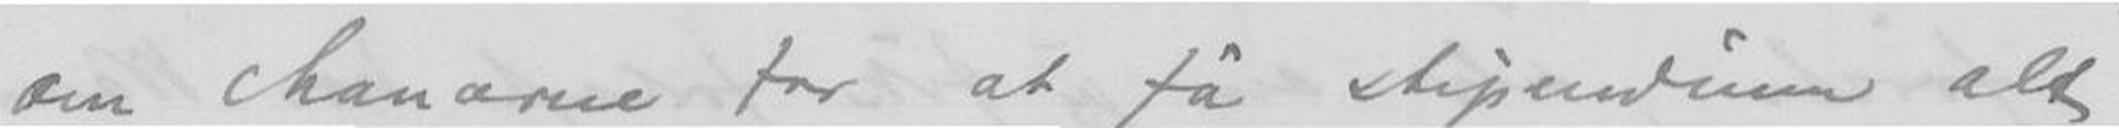om chancerne for at få stipendium alt
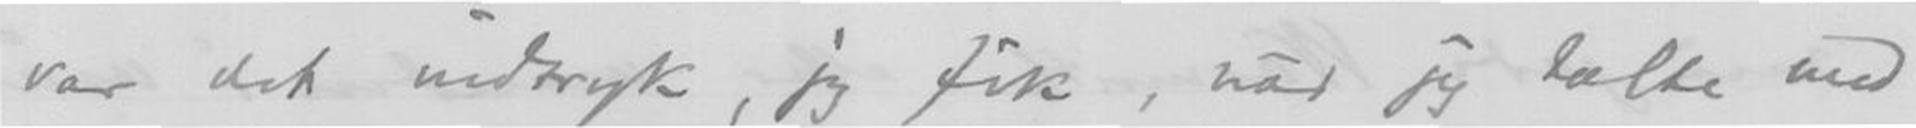var det indtryk, jeg fik, når jeg talte med
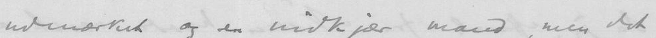udmærket og en nidkjær mand, men det
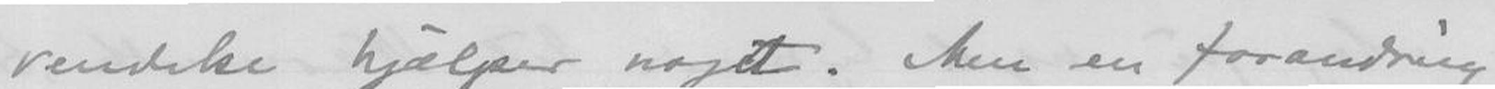vendelse hjælper noget. Men en forandring
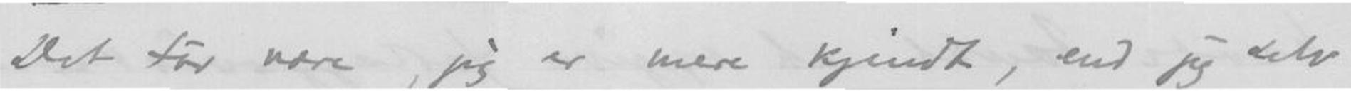Det tør være, jeg er mere kjendt, end jeg selv
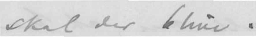skal der blive.
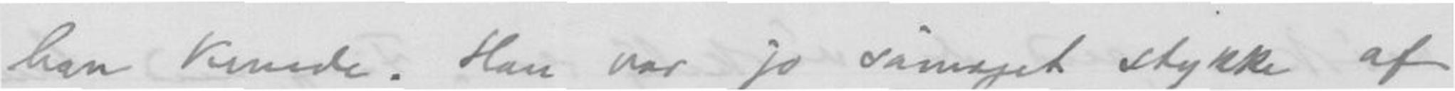han kunde. Han var jo såmeget stykke af
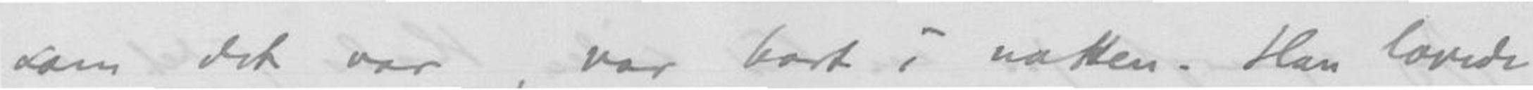som det var, var bort i natten. Han lovede
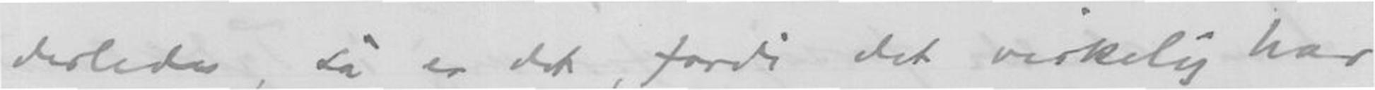derledes, så er det, fordi det virkelig har
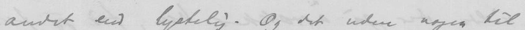andet end lystelig. Og det uden noget til
